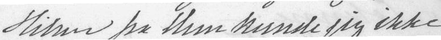Hilsen fra Dem kunde jeg ikke
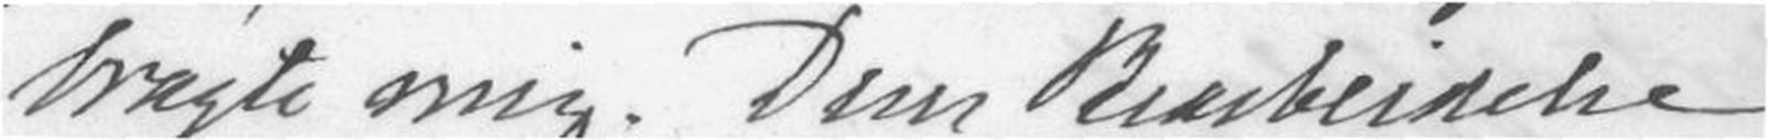bragte mig. Deres Bearbeidelse
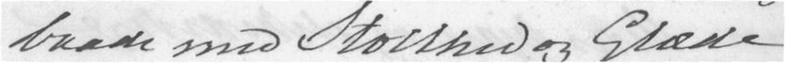baade med Stolthed og Glæde.
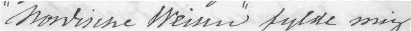"Nordische Weisen" fylde mig
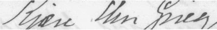Kjære Herr Grieg
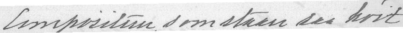Compositum, som staaer saa høit
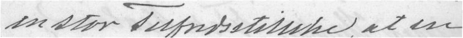en stor Tilfredstillelse, at en
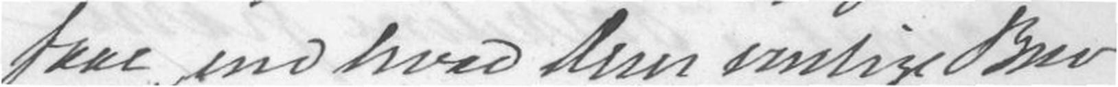faae, end hvad Deres venlige Brev
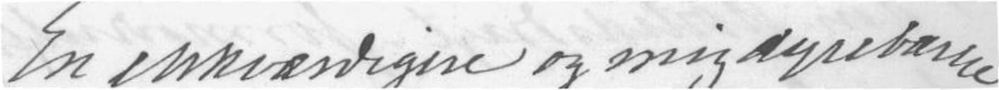En elskværdigne og mig dyrebarne
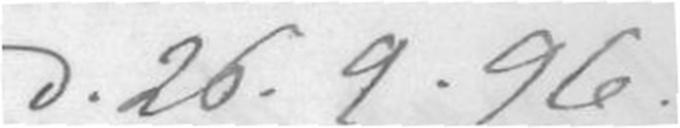D. 26.9.96.
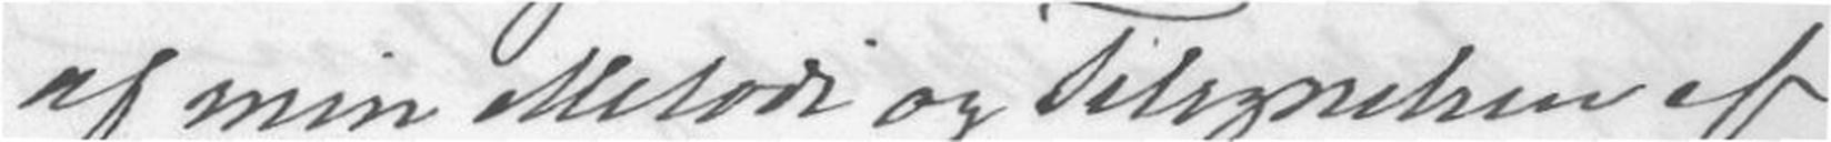af min Melodi og Tilegnelsen af
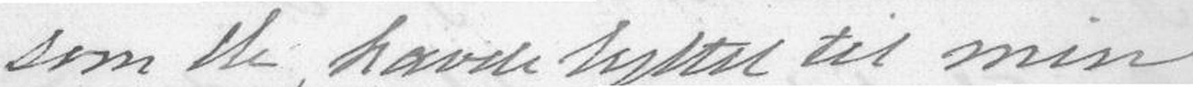som De, havde lyttet til min
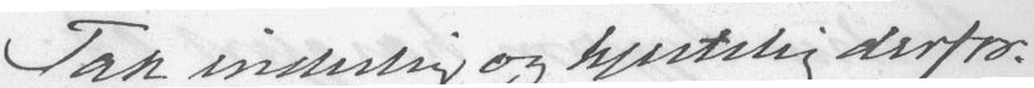Tak inderlig og hjertelig derfor.
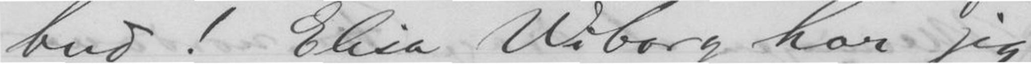bud! Elisa Wiborg har jeg
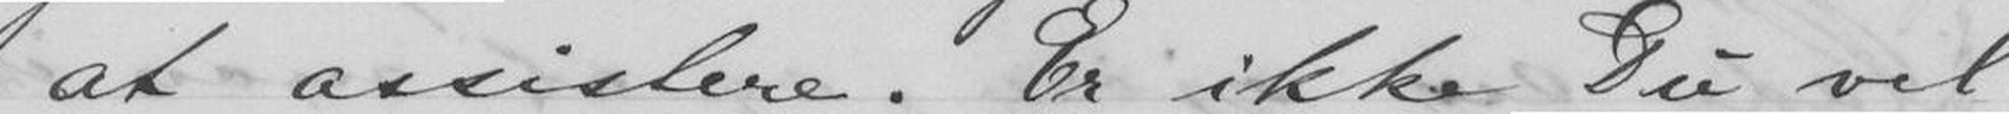at assistere. Er ikke Du vel
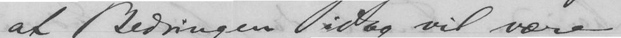at Bedringen idag vil være
